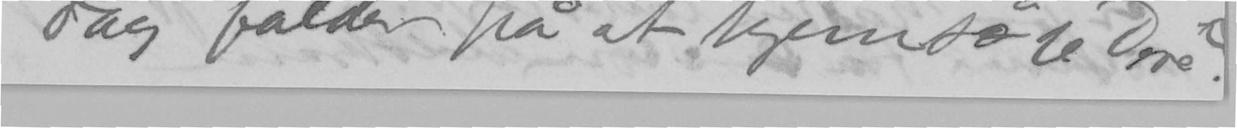dag falder på at hjemsøge Dere?
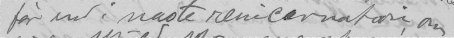før end i næste reincarnation, om
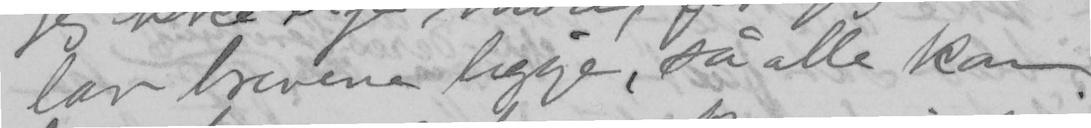lar brevene ligge, så alle kan
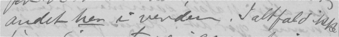andet her i verden. Ialtfald ikke
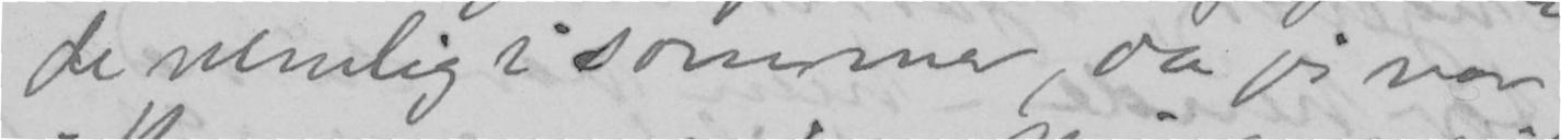de nemlig i sommer, da jeg var
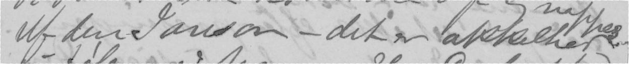Uf den Janson - det er ækkelhed
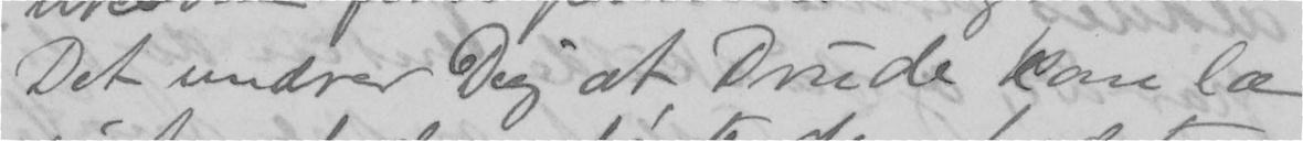Det undrer Dig at Drude kan la
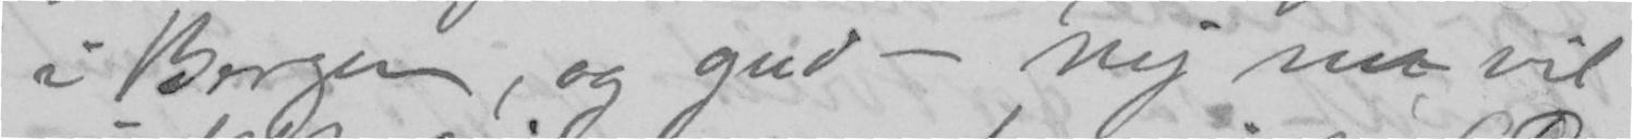i Bergen, og gud - nej nu vil
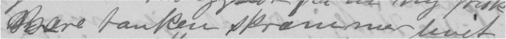Bare tanken skræmmer livet
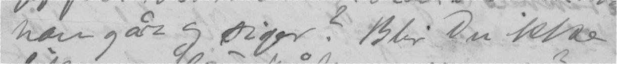han går og siger? Blir Du ikke
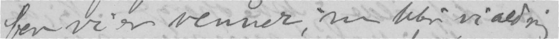for vi er venner, nu blir vi aldrig
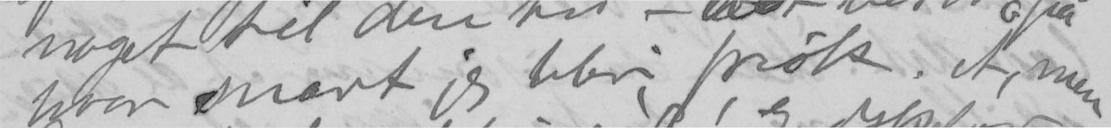hvor snart jeg blir frisk. Å, men
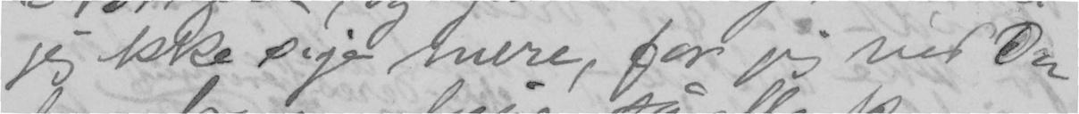jeg ikke sige mere, for jeg ved Du
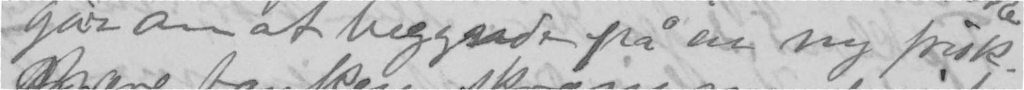går an at begynde på en ny frisk.
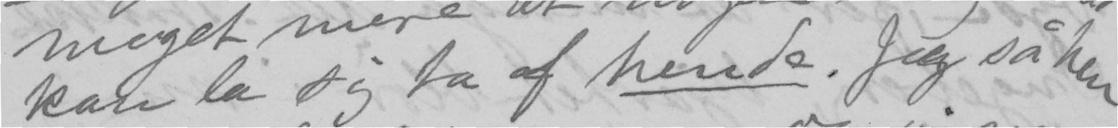kan la sig ta af hende. Jeg så hen
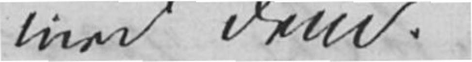med dem.
